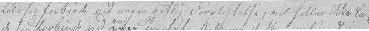lade sig forbinde ved nogen retlig Forpligtelse, vil heller ikke la-
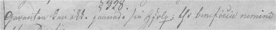Garanten kan ikke paanøde sin Hjælp; thi beneficio nemini
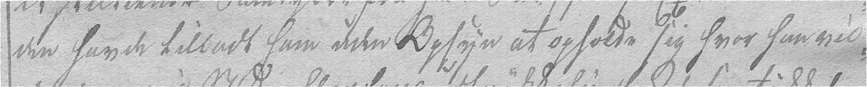den havde tilladt ham uden Opsyn at opholde sig hvor han vil-
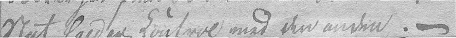Stat holder Control med den anden. –
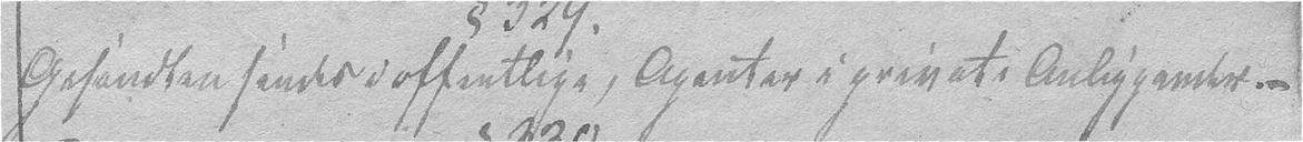Gesandten sendes i offentlige, Agenter i private Anliggender. –
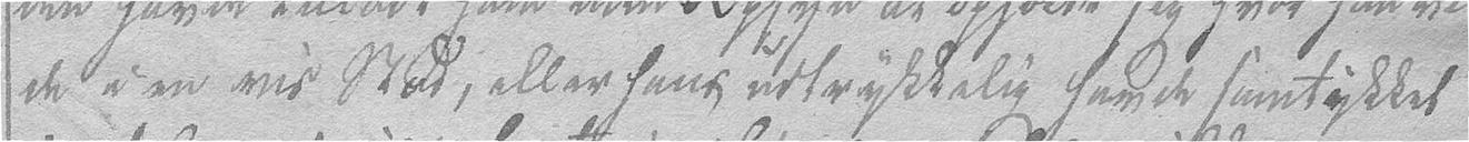de i en vis Stad, eller hans udtrykkelig havde samtykket
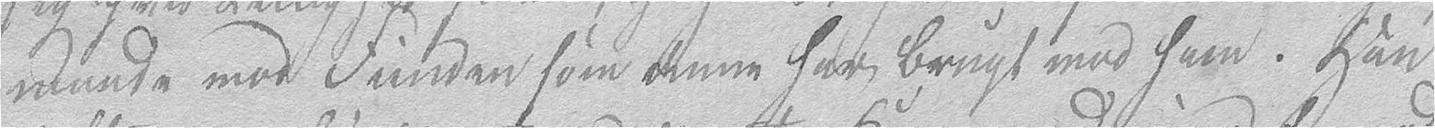maade mod Fienden som denne har brugt mod ham. Han
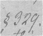§ 329.
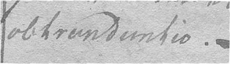obtrundentio.-
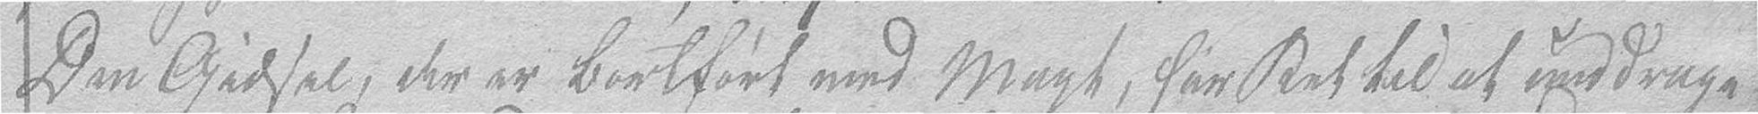Den Gidsel, der er bortført med Magt, har Ret til at unddrage
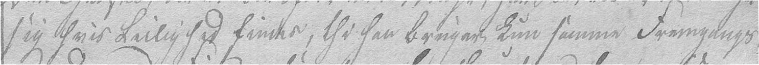sig hvis Leilighed findes, thi han bruger kun samme Fremgangs-
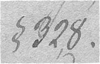§ 328.
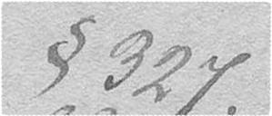§ 327.
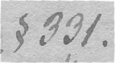§ 331.
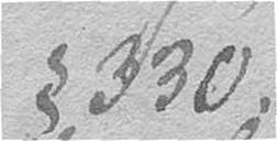§ 330.
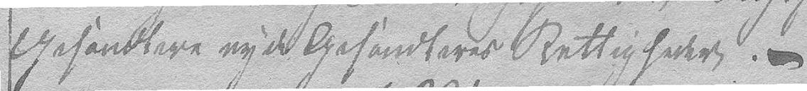Gesandtere nyde Gesandteres Rettigheder. –
